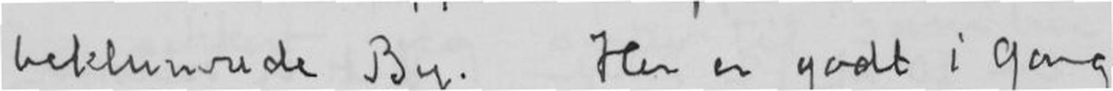beklemmende By. Her er godt igang
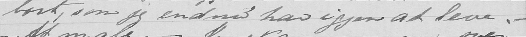bort, som jeg endnu har igjen at leve.-
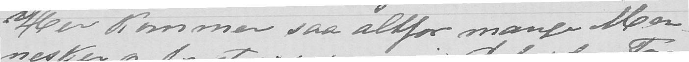Her kommer saa altfor mange Men-
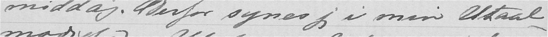middag. Derfor synes jeg i min Utaal-
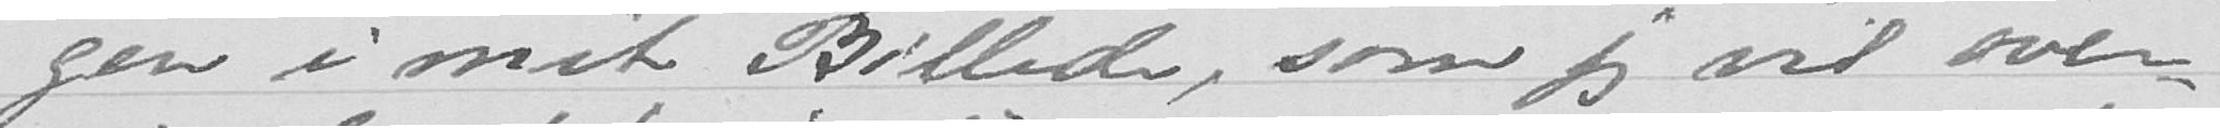gen i mit Billede, som jeg vil over-
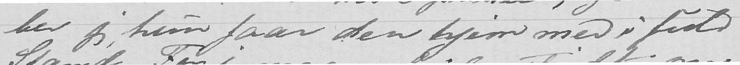ber jeg hun faar den hjem med i fuld
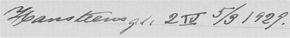Hansteens gt 2 IV 5/3 1929.
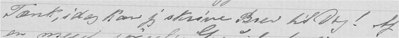Tænk, i dag kan jeg skrive Brev til dig! Af
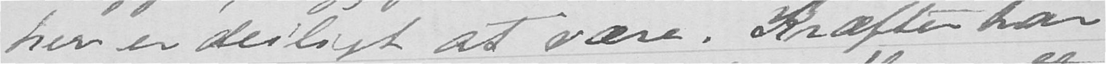her er deiligt at være. Kræfter har
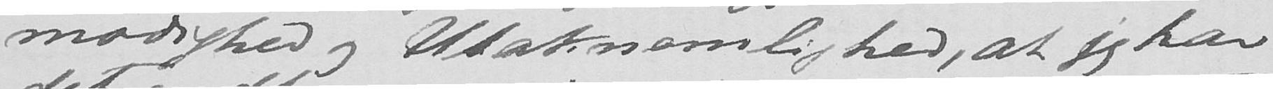modighed og Utaknemlighed, at jeg har
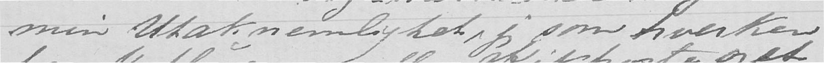min Utaknemlighet, jeg som hverken
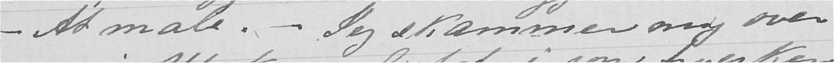- At male. - Jeg skammer mig over
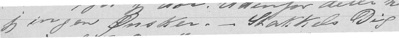jeg ingen Ønsker. - Stakkels Dig
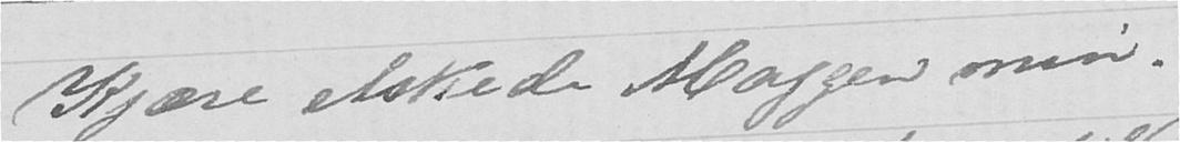Kjære elskede Maggen min.
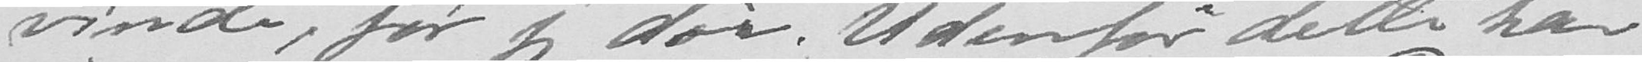vinde, før jeg dør. Udenfor dette har
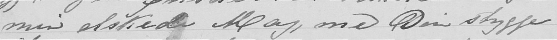men elskede Mag, med Din stygge
